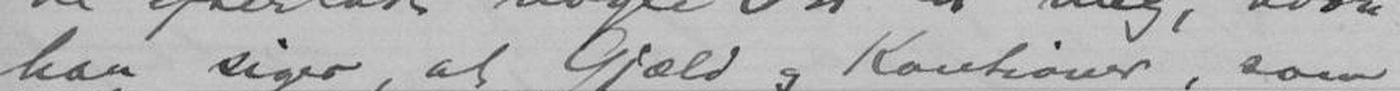han siger, at Gjæld og Kontioner, som
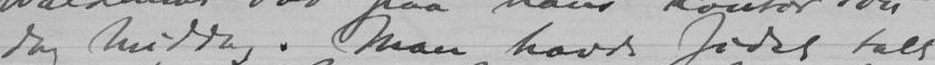dag Middag. Man havde sidst talt
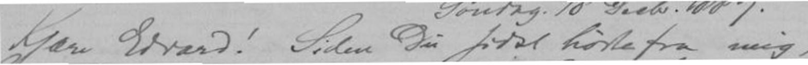Kjære Edvard!Siden Du sidst hørte fra mig,
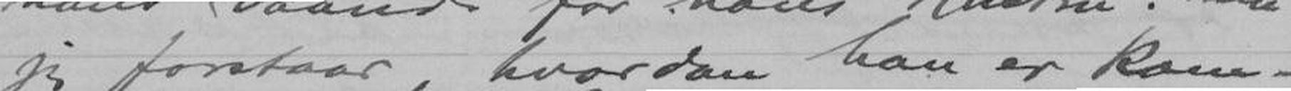jeg forstaar, hvordan han er kom-
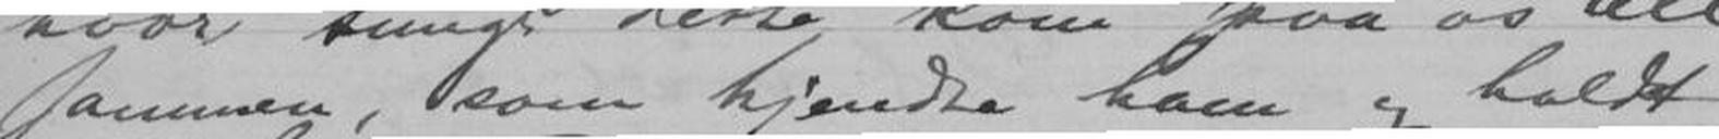sammen, som kjendte ham og holdt
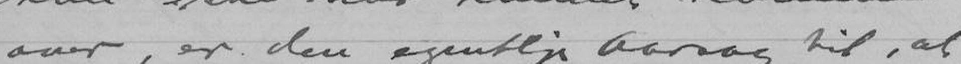over, er den egentlige Aarsag til, at
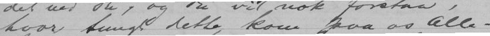hvor tungt dette kom paa os Alle-
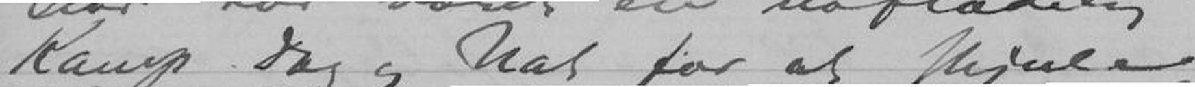Kamp Dag og Nat for at skjule
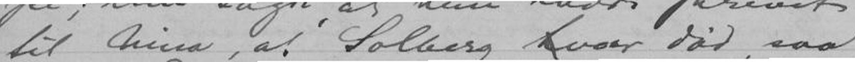til Nina, at Solberg hvar Død, saa
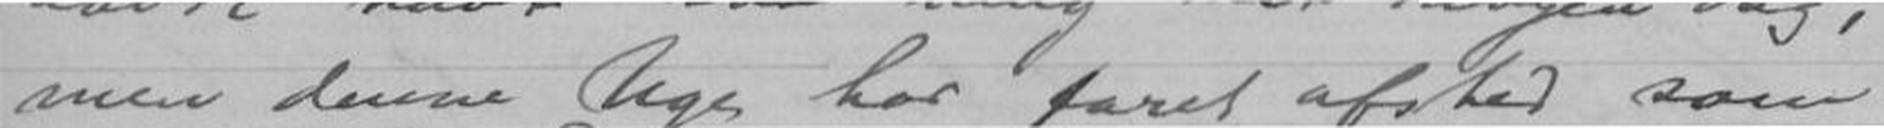men denne Uge har faret afsted som
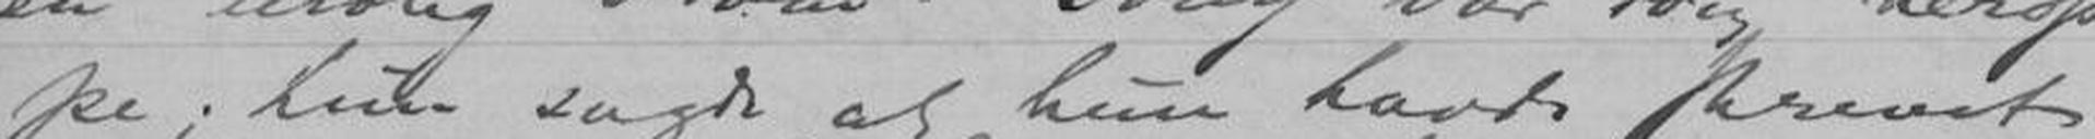pe; hun sagde, at hun havde skrevet
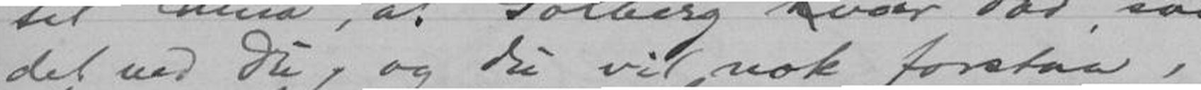det ved Du, og Du vil nok forstaa,
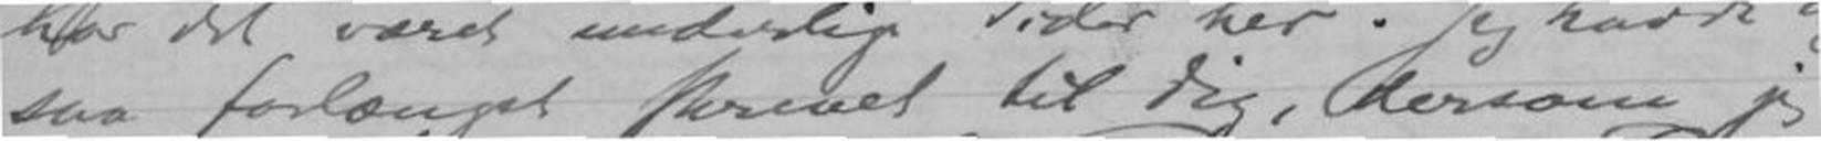saa forlængst skrevet til Dig, dersom jeg
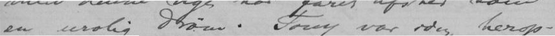en urolig Drøm. Tony var idag herop-
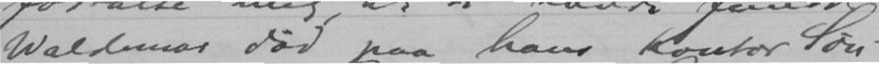Waldemar Død paa hans Kontor Søn-
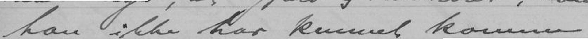han ikke har kunnet komme
